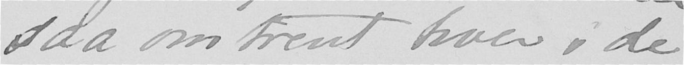saa omtrent hver i de
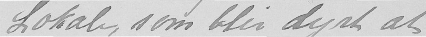Lokale, som blir dyrt at
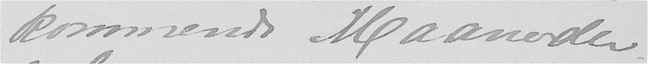kommende Maaneder
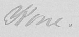Kone.
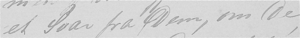et Svar fra Dem, om De
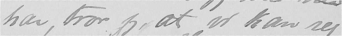har, tror jeg at vi kan reg-
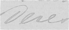Deres
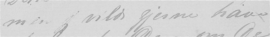men jeg vilde gjerne have
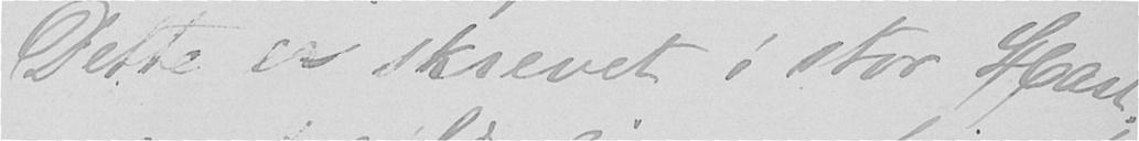Dette er skrevet i stor Hast,
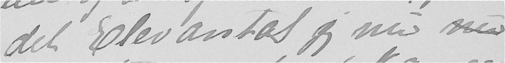det Elevantal jeg nu
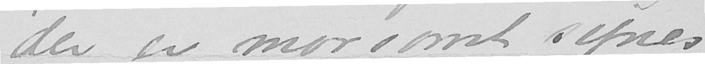der er morsomt synes
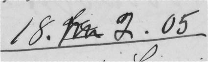18. 2.05
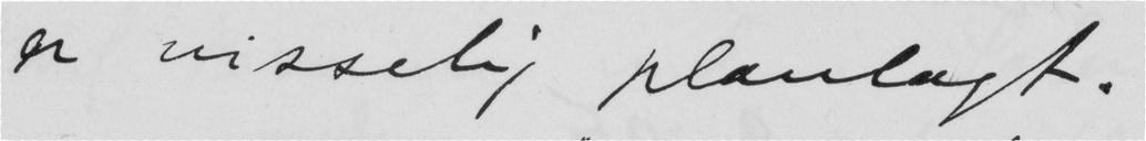er visselig planlagt.
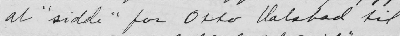at "sidde" for Otto Valstad til
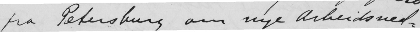fra Petersburg om nye Arbeidsned-
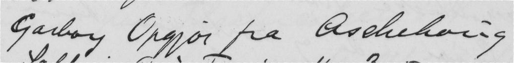Garborg Opgjør fra Aschehoug
